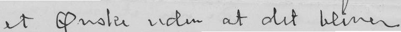et Ønske uden at det bliver
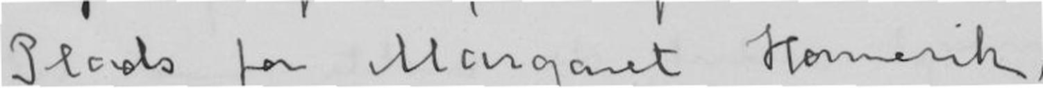Plads for Margaret Homervik,
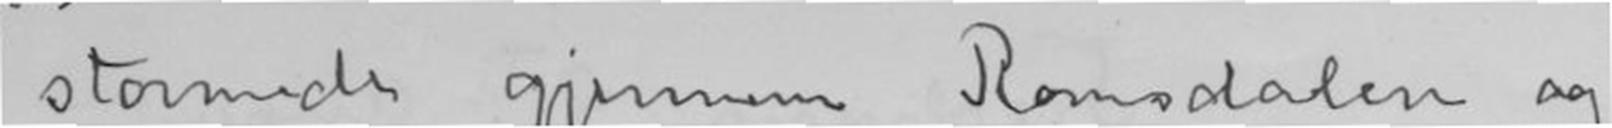Stormede gjennem Romsdalen og
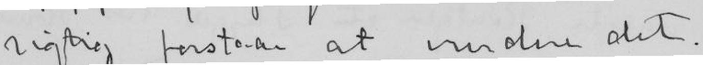vigtige forstaar at vurdere det.
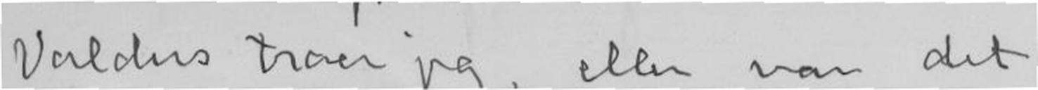Valdus troer jeg, eller var det
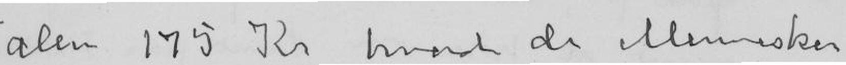alene 175 kr, hvad de Mennesker
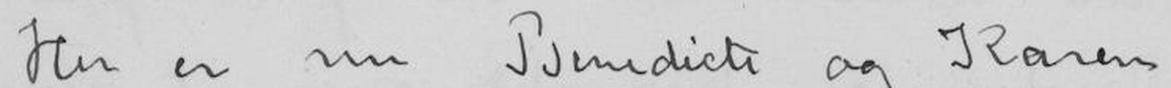Her er nu Benedicte og Karen.
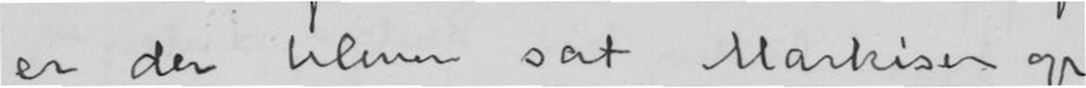er der bleven sat Markiser op,
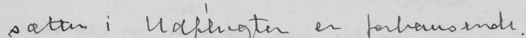sætter i Udflugter er forbausende.
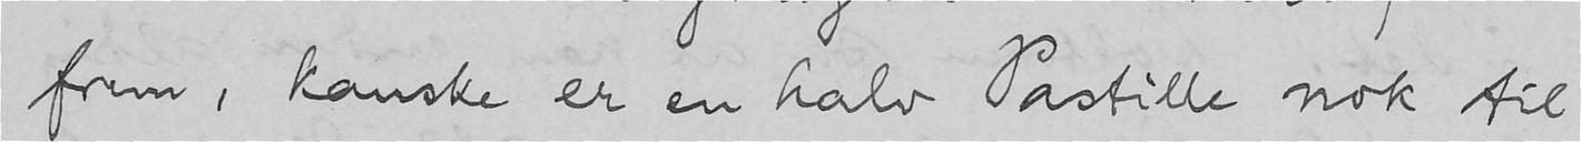frem, kanske er en halv Pastille nok til
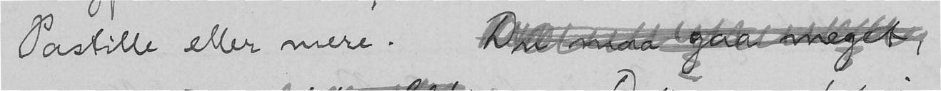Pastille eller mere. Du maa gaa meget,
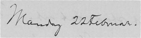Mandag 22 Februar.
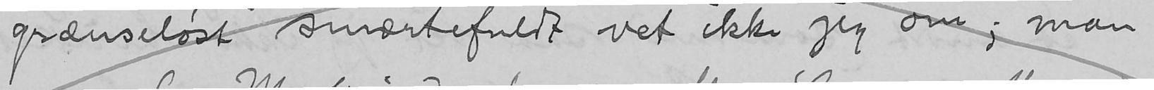grænseløst smærtefuldt vet ikke jeg om; man
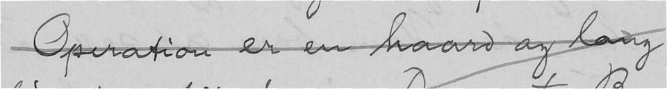Operation er en haard og lang
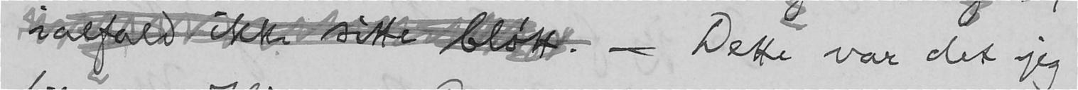ialfald ikke sitte blødt. - Dette var det jeg
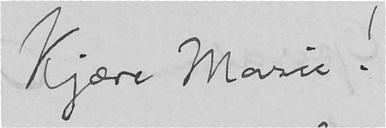Kjære Marie!
